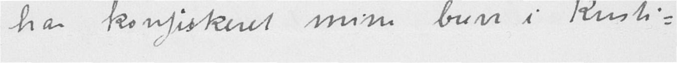har konfiskeret mine breve i Kristi-
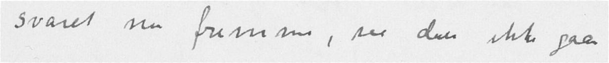svaret nu fremme, saa du ikke gaar
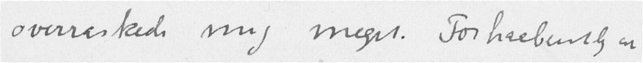overraskede mig meget. Forhaabentlig er
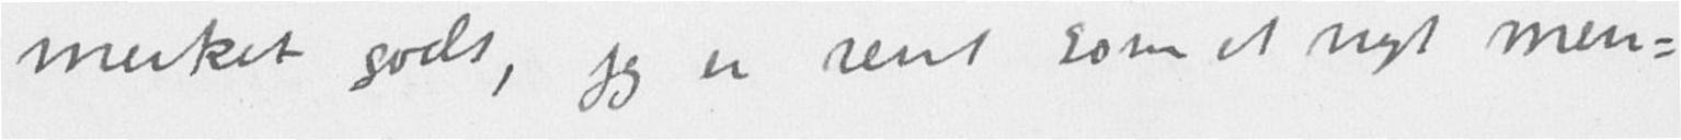merket godt, jeg er rent som et nyt men-
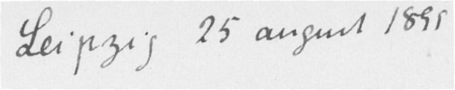Leipzig 25 august 1899
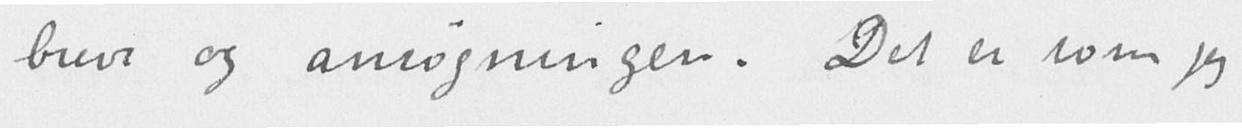breve og ansøgninger. Det er som jeg
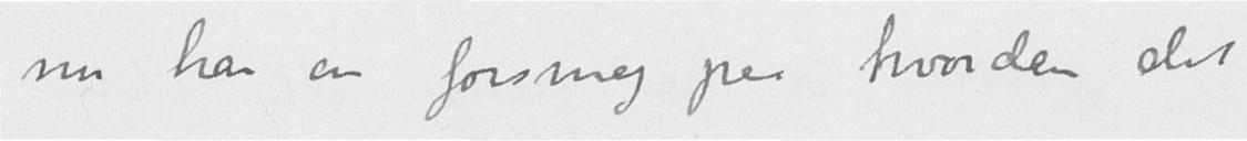nu har en forsmag paa hvordan det
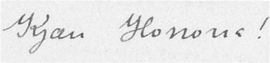Kjære Honoria!
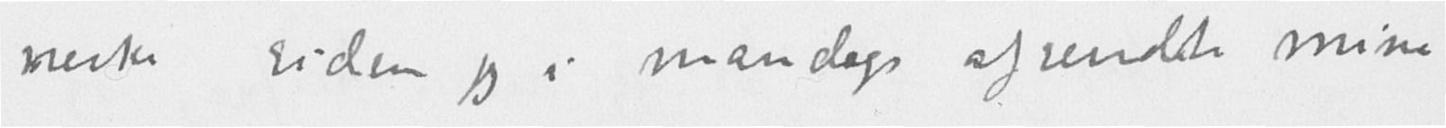neske siden jeg i mandags afsendte mine
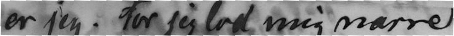er jeg. For jeg lod mig narre
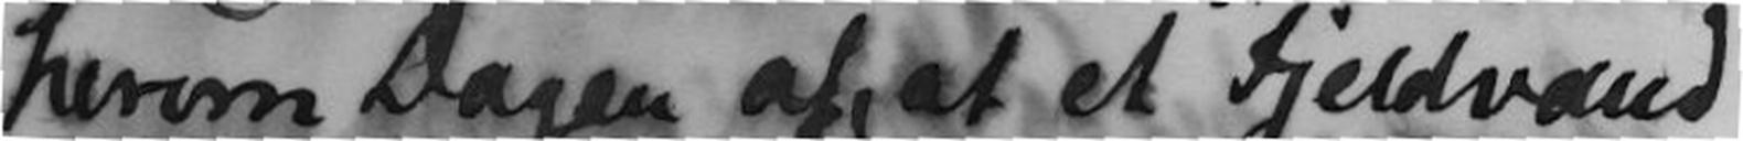herom Dagen af, at et Fjeldvand
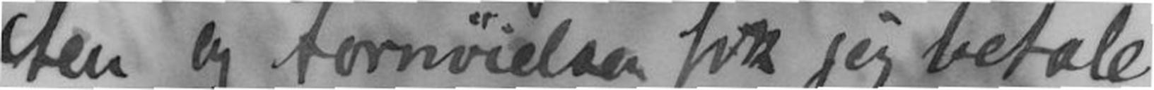ten og Fornøielsen fik jeg betale
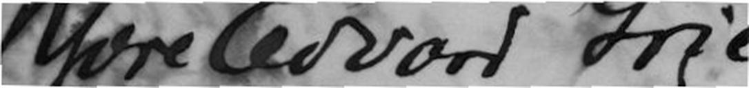Kjære Edvard Grieg,
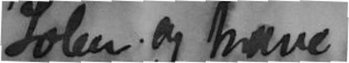Solen. Og have
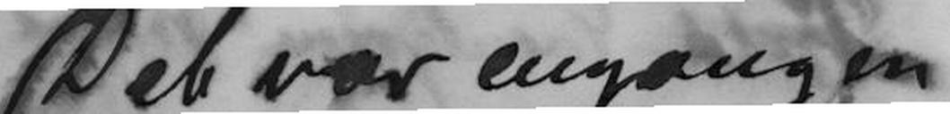Det var engang en
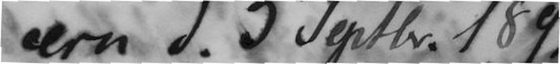ern d. 5 Septbr. 1892.
